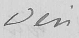Din
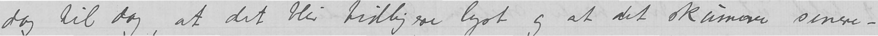dag til dag, at det blir tidligere lyst og at det skumrer senere –
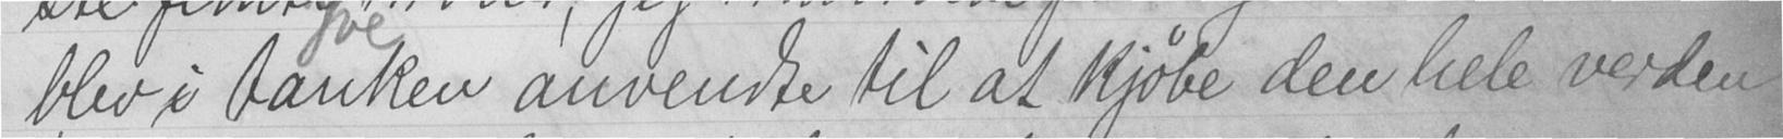blev i tanken anvendte til at kjøbe den hele verden
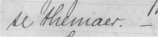se themaer. -
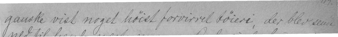ganske vist noget høist forvirret tøieri, der blev sendt
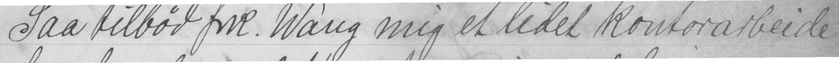Saa tilbød frk. Wang mig et lidet kontorarbeide
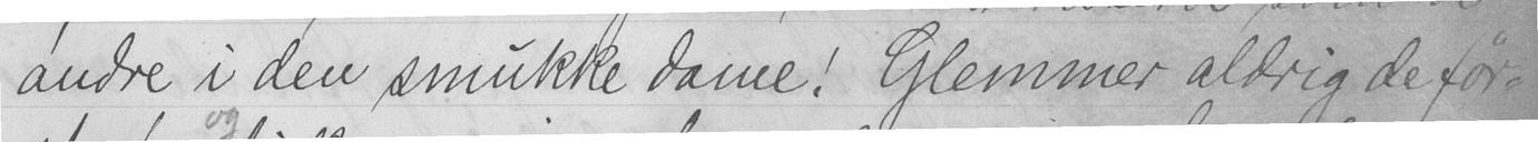andre i den smukke dame! Glemmer aldrig de før-
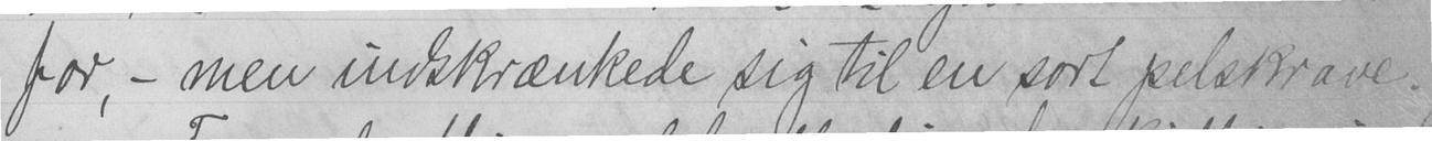for, - men indskrænkede sig til en sort pelskrave.
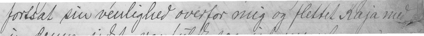fortsat sin venlighed overfor mig og flettet Kaja med
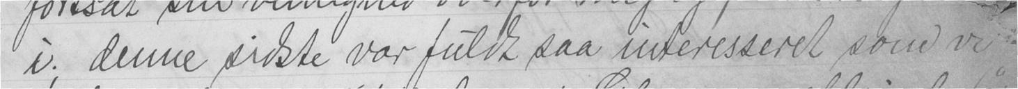i; denne sidste var fuldt saa interesseret som vi
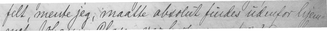felt, mente jeg, maatte absolut findes udenfor hjem-
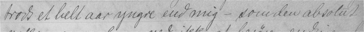trods et helt aar yngre end mig - som den absolut
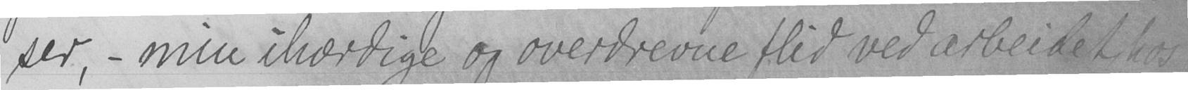ser, - min ihærdige og overdrevne flid ved arbeidet hos
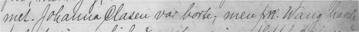met. Johanna Clasen var borte; men frk. Wang havde
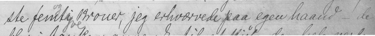ste femogtyve kroner, jeg erhværvede paa egen haand - de
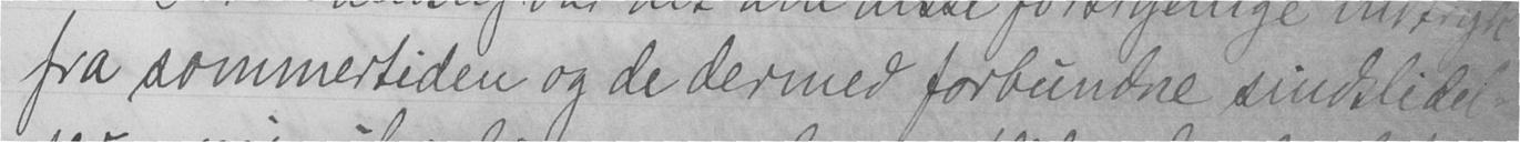fra sommertiden og de dermed forbundne sindslidel-
